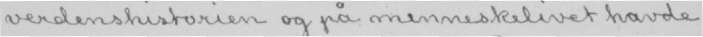verdenshistorien og på menneskelivet havde
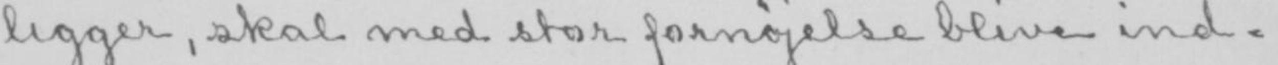ligger, skal med stor fornøjelse blive ind-
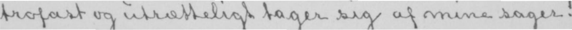trofast og utrætteligt tager sig af mine sager!
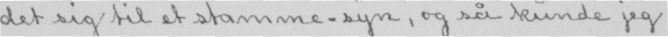det sig til et stamme-syn, og så kunde jeg
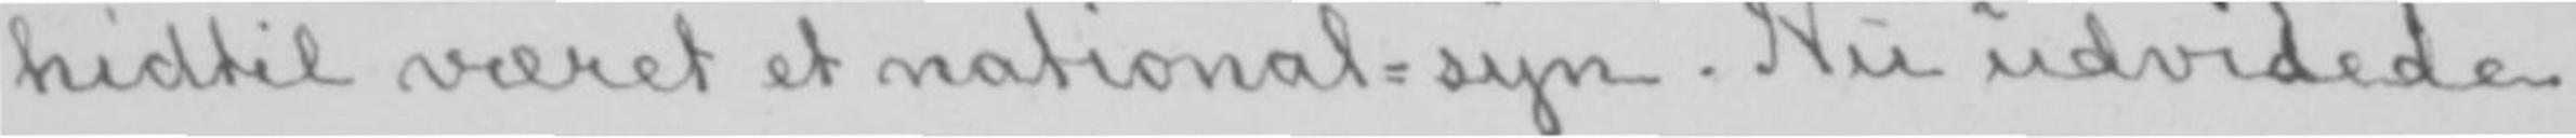hidtil været et national-syn. Nu udvidede
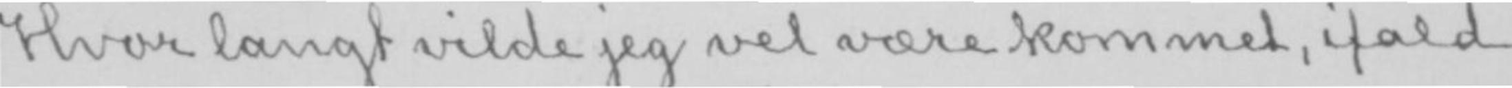Hvor langt vilde jeg vel være kommet, ifald
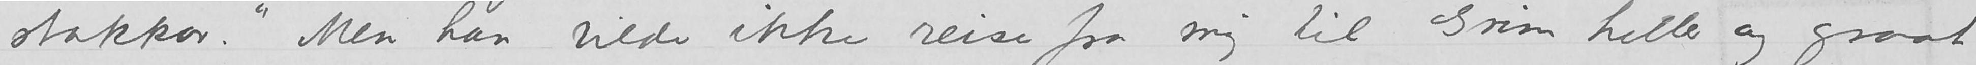stakkar.» Men han vilde ikke reise fra mig til Grim heller og graat
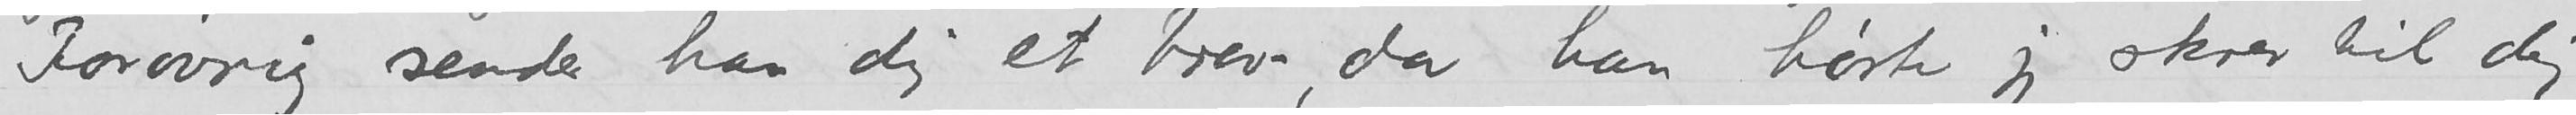Forøvrig sender han dig et brev, da han hørte jeg skrev til dig
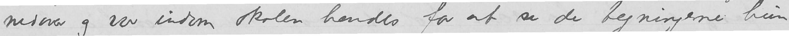nedover og var indom skolen hendes for at se de tegningene hun
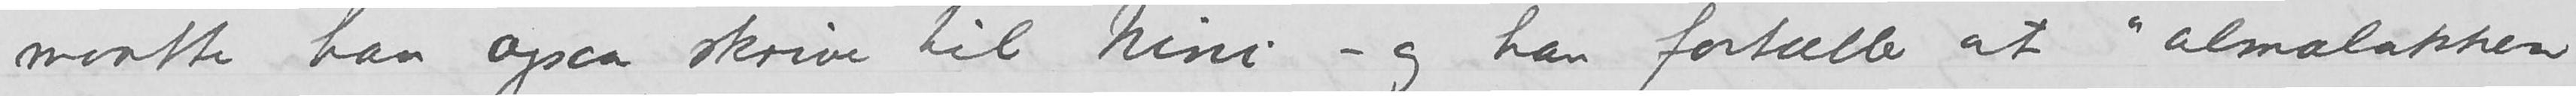maatte han ogsaa skrive til Nini – og han fortæller at «almalakken
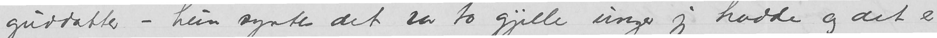guddatter – hun syntes det var to gjille unger jeg hadde og det er
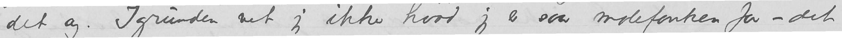det og. Igrunden vet jeg ikke hvad jeg er saa molefonken for – det
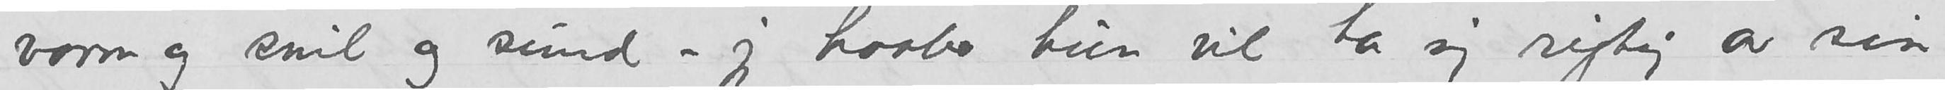saa varm og snil og sund – jeg haaber hun vil ta sig rigtig av sin
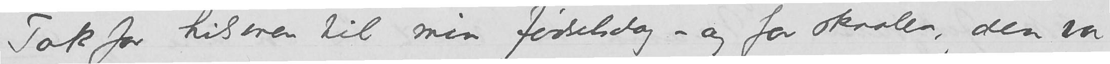Tak for hilsenen til min fødselsdag – og for skaalen, den var
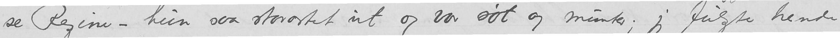se Regine – hun saa storartet ut og var søt og munter; jeg fulgte hende
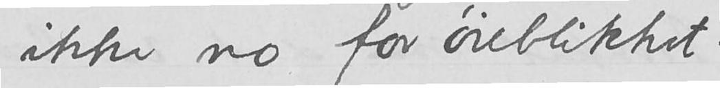ikke no for øieblikket.
